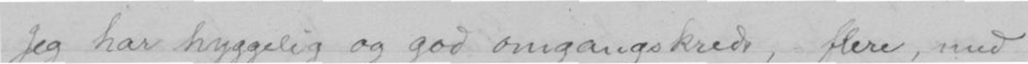Jeg har hyggelig og god omkreds, flere med
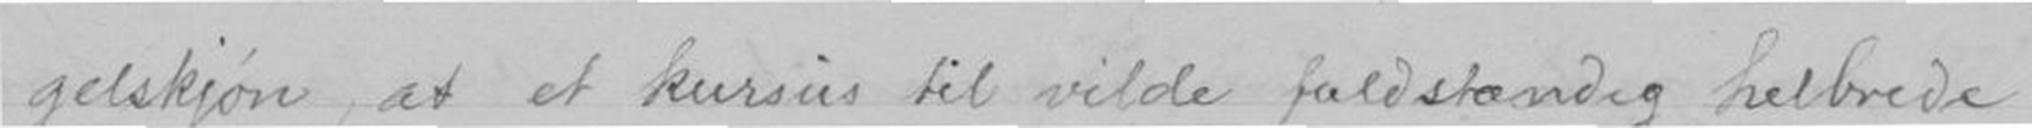gelskjøn at et kursus til vilde fulstændig helbrede
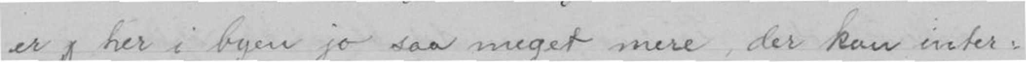er her i byen jo saa meget mere, der kan inter-
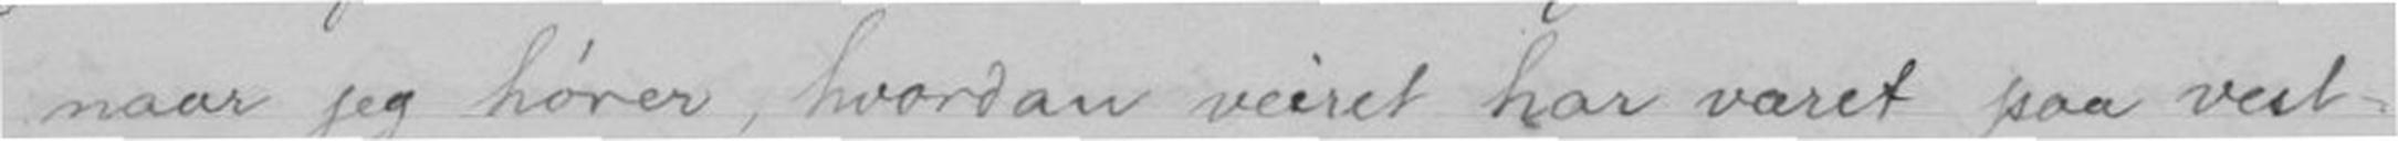naar jeg hører, hvordan veiret har været paa vest-
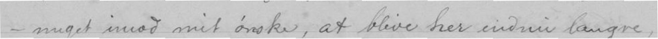- meget imod mit ønske, at blive her endnu længre,
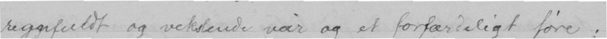regnfuldt og vekslende veir og et forfærdiligt føre;
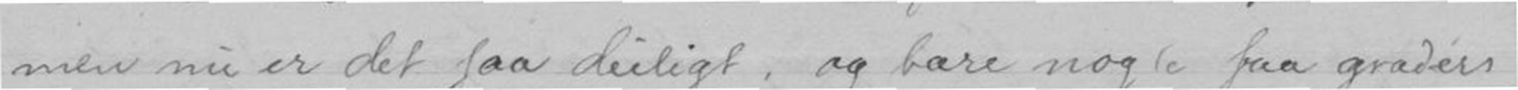men nu er det saa deiligt og bare nogle faa graders
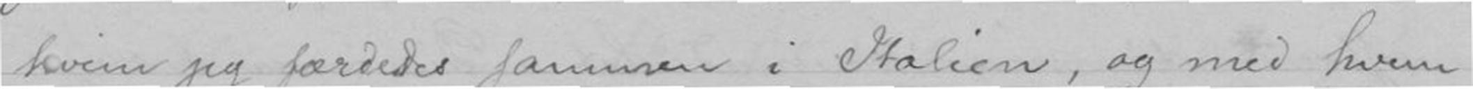hvem jeg færdes sammen i Italien, og med hvem
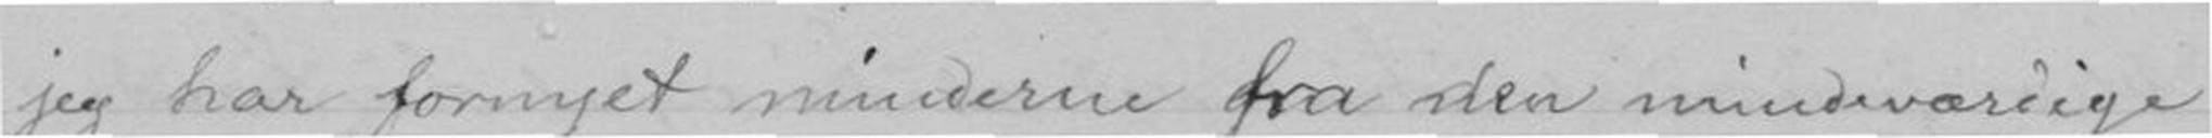jeg har fornyet minderne fra den mindeværdige
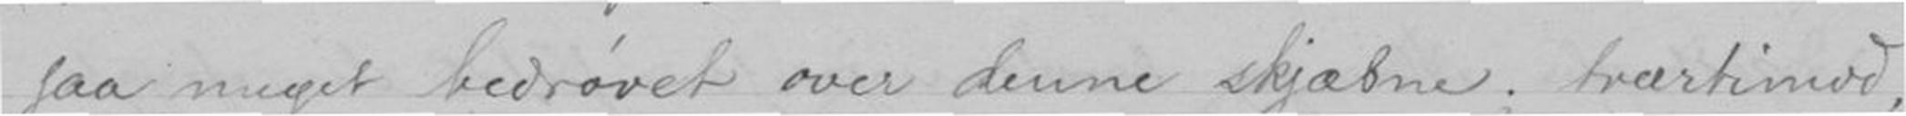saa meget bedrøvet over denne skjæbne; tvæerimod,
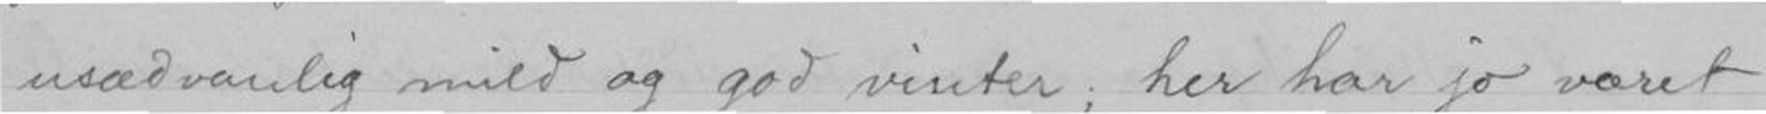usædvanlig mild og god vinter; her har jo været
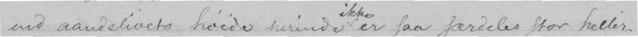end aandslivets høiede herinde er saa særdeles stor heller.
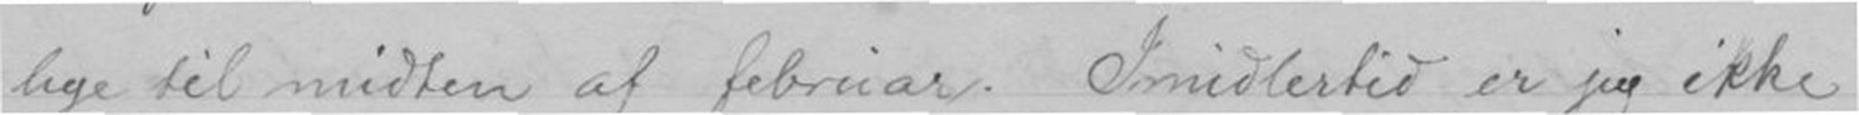lige til midten af februar. Imidlertid er jeg ikke
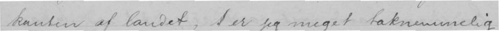kanten af landet er jeg meget taknemmelig
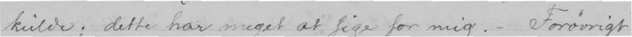kulde; dette har meget at sige for mig. - Forøvrigt
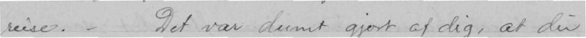reise. - Det var dumt gjort af dig, at du
